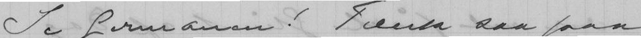Se Germanen! Tenk saa paa
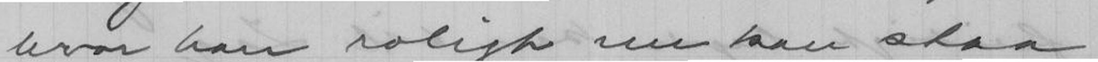hvor han roligt nu kan staa
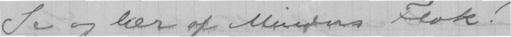Se og lær af Minders Flok!
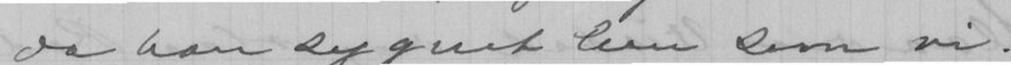da han sygnet hen som vi.
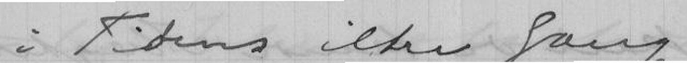som der i Tidens iltre Gang
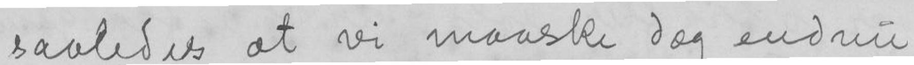saaledes at vi maaske dog endnu
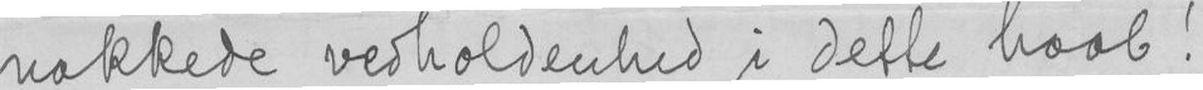nakkede vedholdenhed i dette haab!
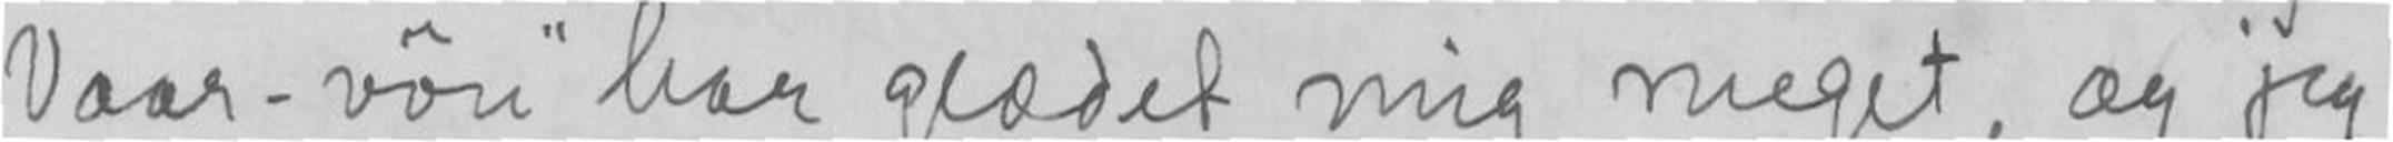"Vaar-vön" har glædet mig meget, og jeg
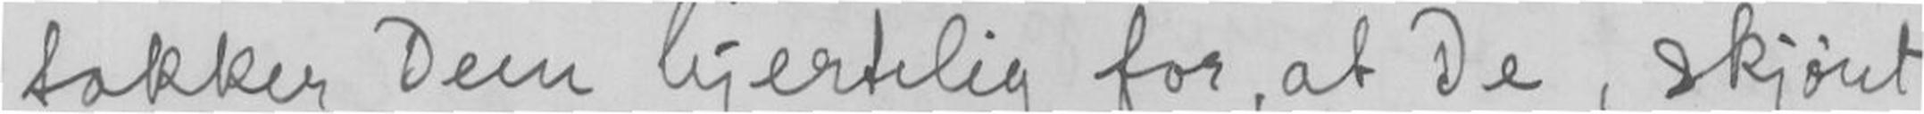takker Dem hjertelig for, at De, skjønt
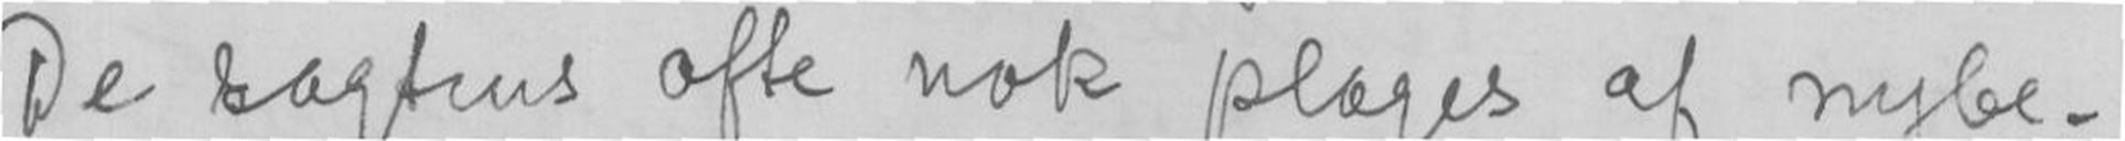De sagtens ofte nok plages af nybe-
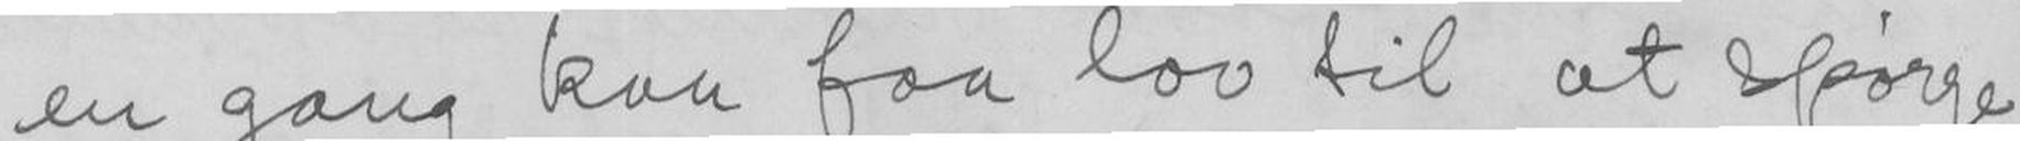en gang kan faa lov til at spørge
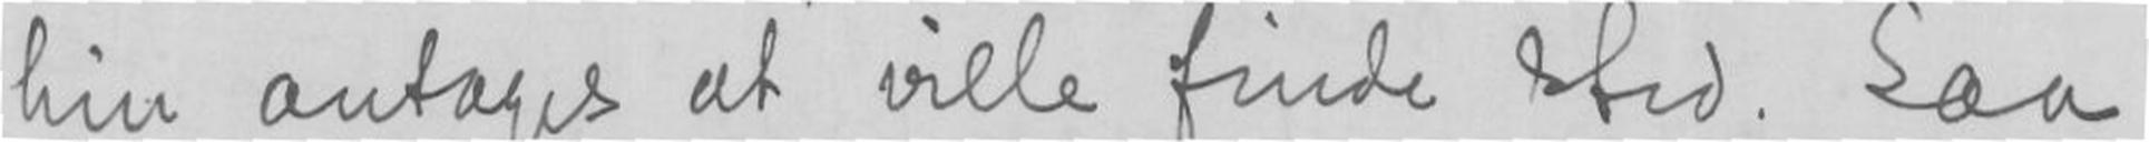hin antages at ville finde sted. Saa
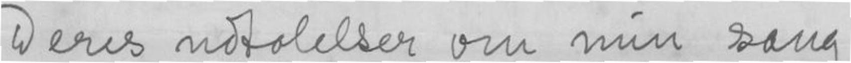Deres udtalelser om min sang
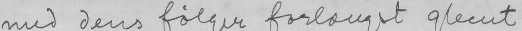med dens følger forlængst glemt
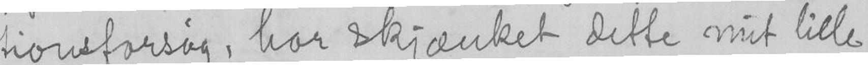tionsforsøg, har skjænket dette mit lille
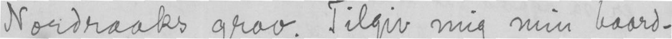Nordraaks grav. Tilgiv mig min haard-
